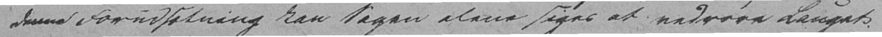denne Forudsætning kan Sagen alene siges at vedrøre Lauget.
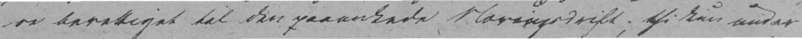re berettiget til den paaankede Næringsdrift; thi kun under
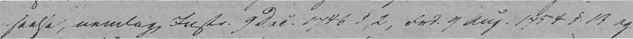seelse, nemlig Instr. 9 Dec. 1746 § 2, Frd. 9 Aug. 1754 § 13 og
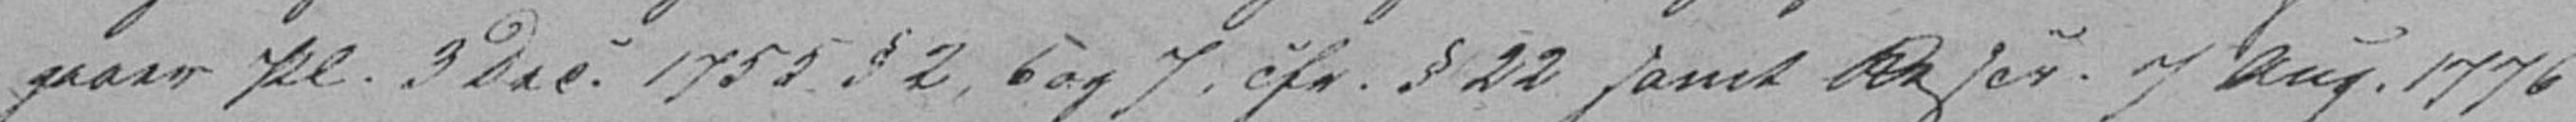gaaer Pl. 3 Dec. 1755 § 2, 5 og 7 cfr. § 22 samt Rescr. 7 Aug. 1776
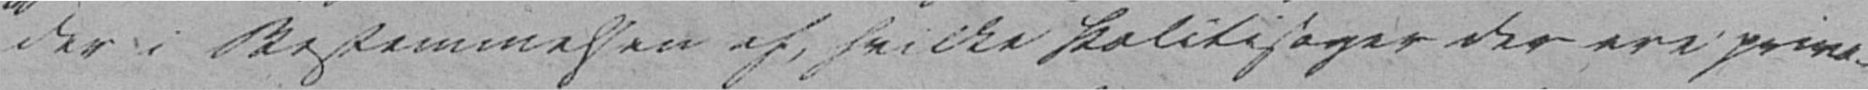der i Bestemmelsen af, hvilke Politisager der ere priva-
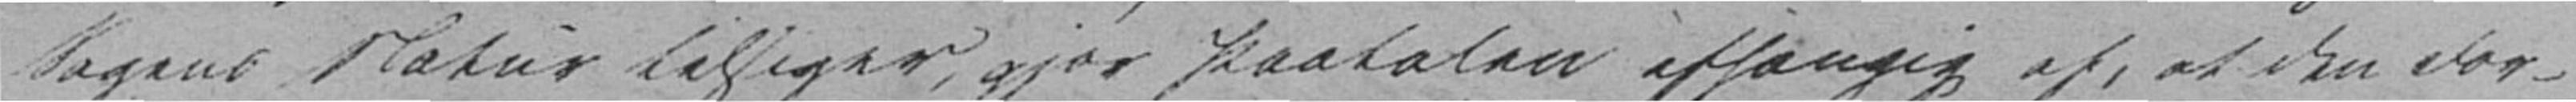Sagens Natur tilsiger, gjør Paatalen afhængig af, at den For-
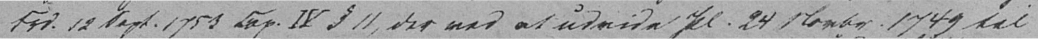Frd. 12 Sept. 1753 Cap. IV § 11, der ved at udvide Pl. 24 Novbr. 1749 til
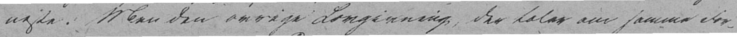neste. Men den øvrige Lovgivning, der taler om samme For-
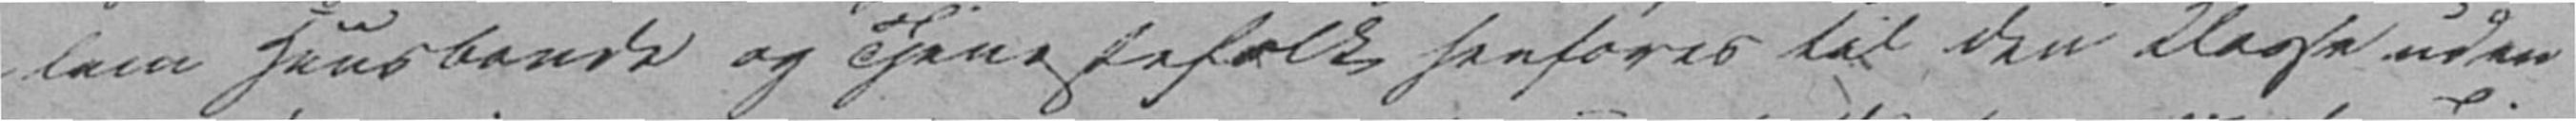lem Huusbonde og Tjenestefolk henføres til den Klasse uden
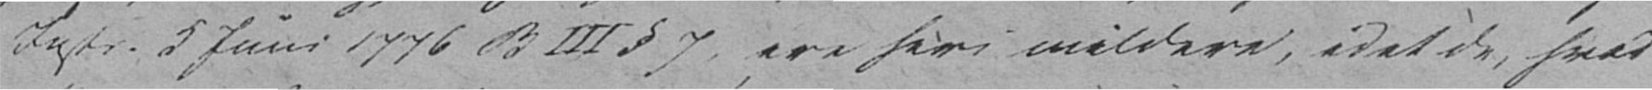Instr. 5 Juni 1776 B III § 7, ere heri mildere, idet de, hvad
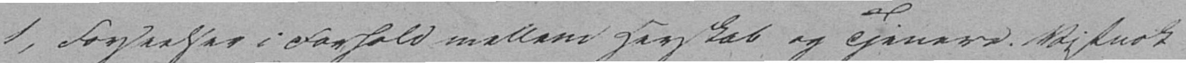1, Forseelser i Forhold mellem Herskab og Tjenere. Vistnok
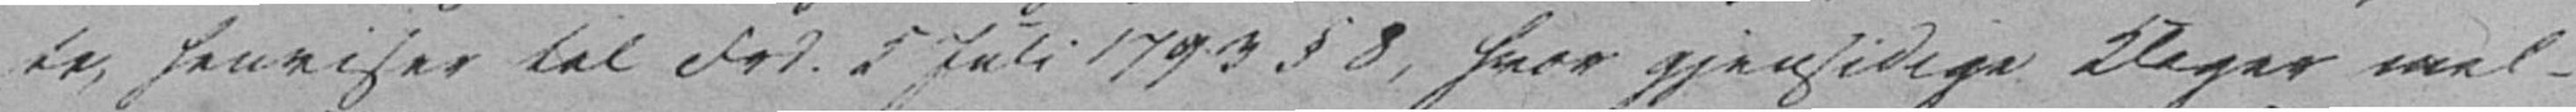te, henviser til Frd. 5 Juli 1793 § 8, hvor gjensidige Klager mel-
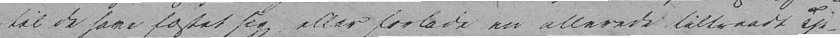til de have fæstet sig, eller forlade en allerede tiltraadt Tje-
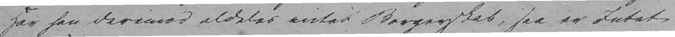Har han derimod aldeles intet Borgerskab, saa er Intet
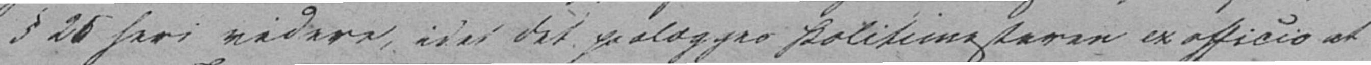§ 25 heri videre, idet det paalægges Politimesteren ex officio at
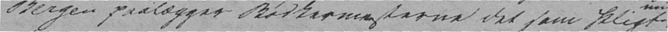Bergen paalægger Bødkermesterne det som Pligt
